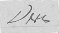Deres
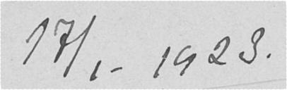17/1-1923.
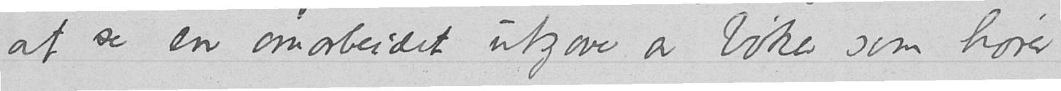at se en omarbeidet utgave av bøker som hører
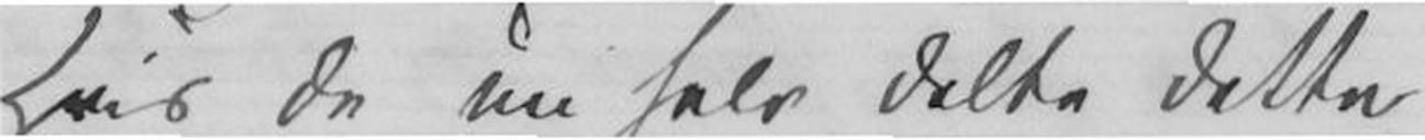Hvis de nu selv delte dette
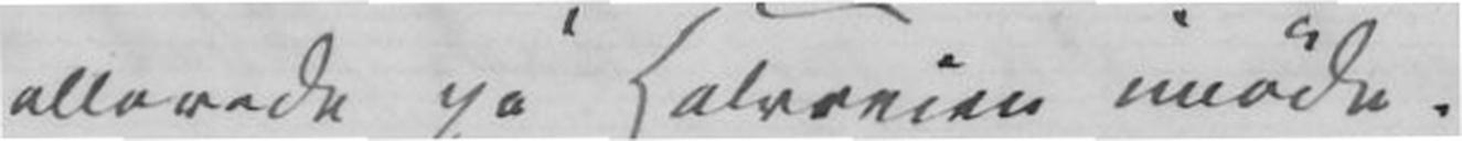allerede på halveien imøde.
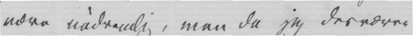være nødvendig, men da jeg desværre ikke
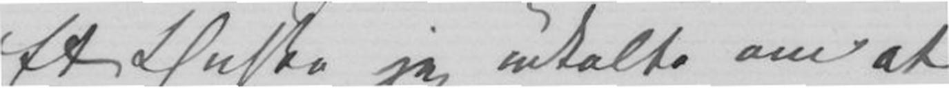Et Ønske jeg udtalte om at
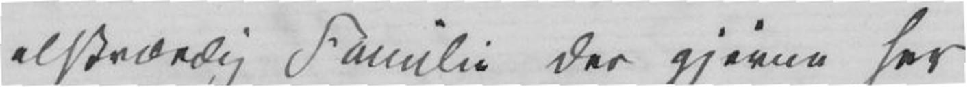elskværdig Familie der gjerne har
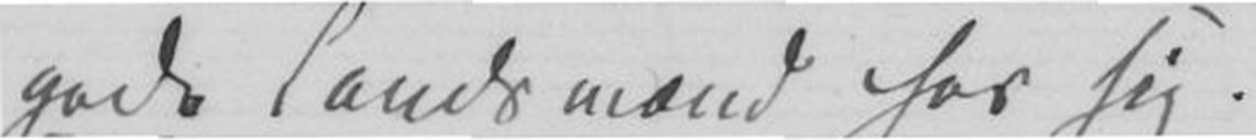gode landsmænd hos sig.
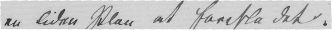en liden plan at foreslå det.
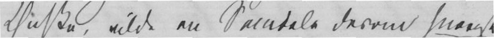Ønske, vilde en Samtale derom smagt
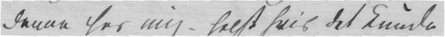denne hos mig - helst hvis det kunde
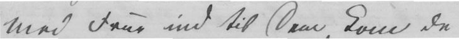med Frue ind til Dem. Kom De
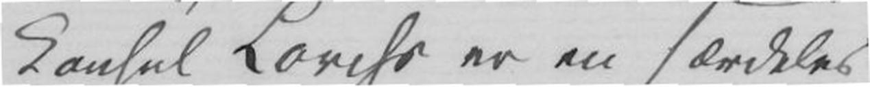Konsul Lorchs er en særdeles
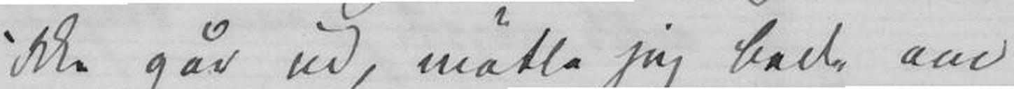går ud, måtte jeg bede om
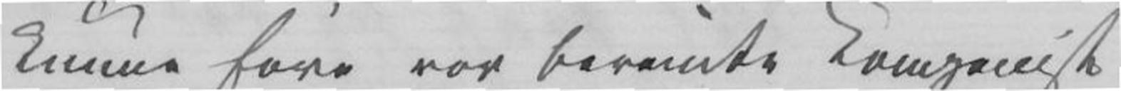kunne føre vor berømte Komponist
